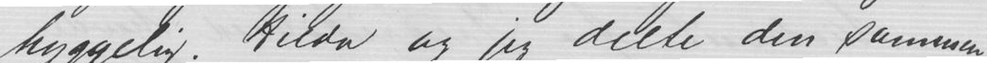hyggelig. Hilde og jeg delte den sammen,
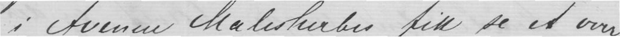i Aveneu Maleshurbes fik se et over-
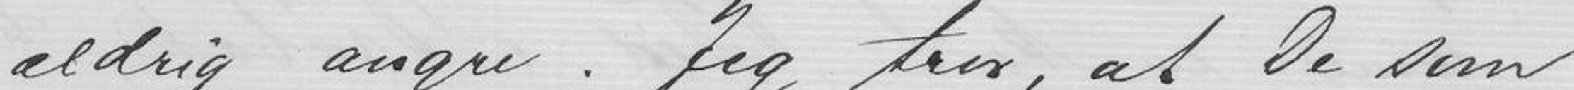aldrig angre. Jeg tror, at De som
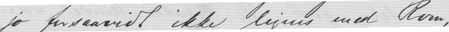jo forsaavidt ikke lignes med Rom,
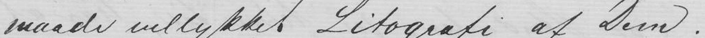maade vellykket Litografi af Dem.
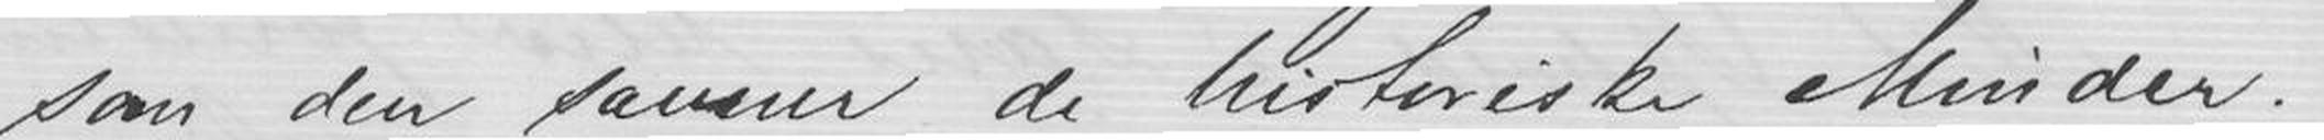som den savner de historiske Minder.
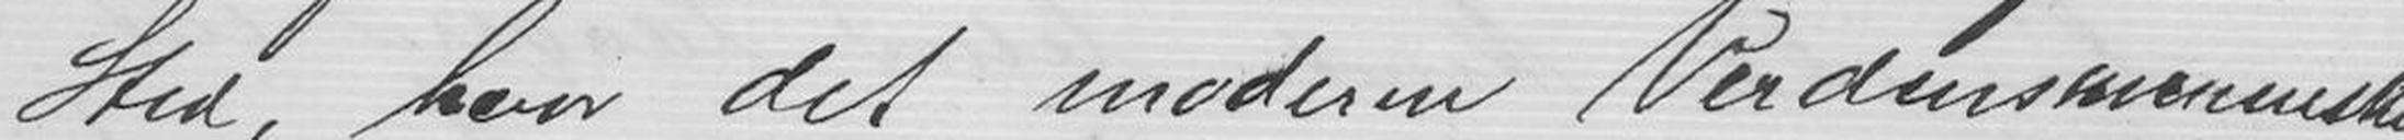Sted, hvor det moderne Verdenmænneske
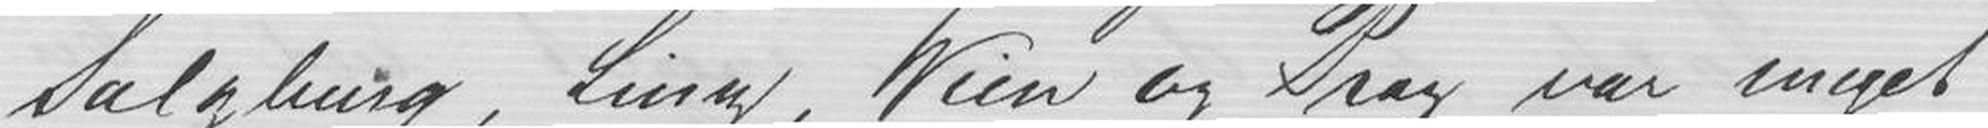Salzburg, Linz. Wien og Prag var meget
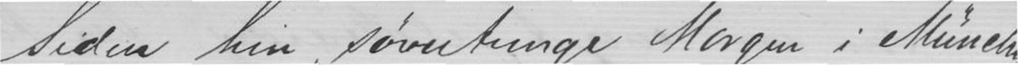Siden hin "søvntunge Morgen i München
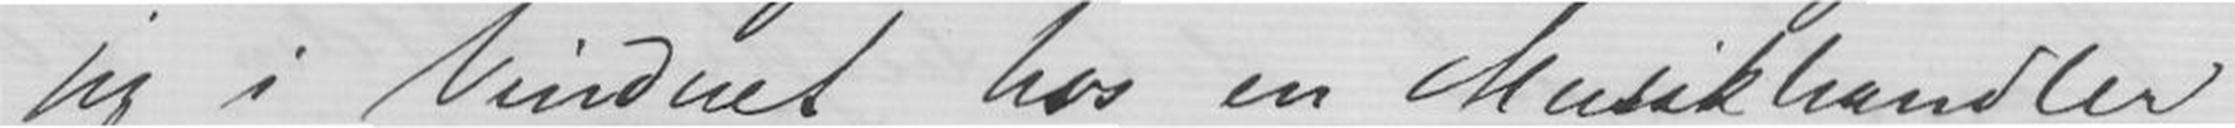jeg i Vinduet hos en Musikhandler
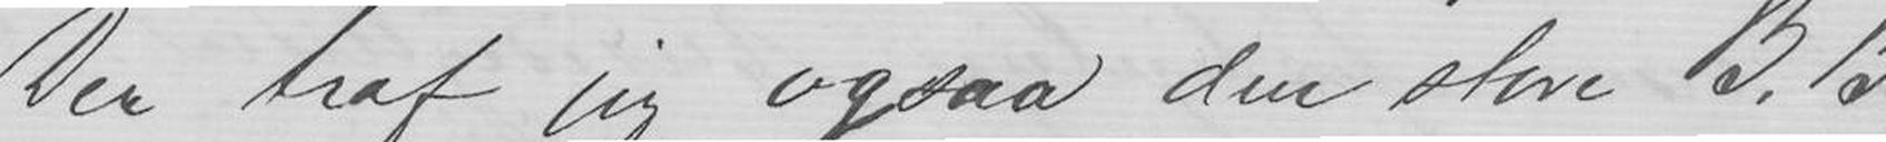Der traf jeg ogsaa den store B.B.
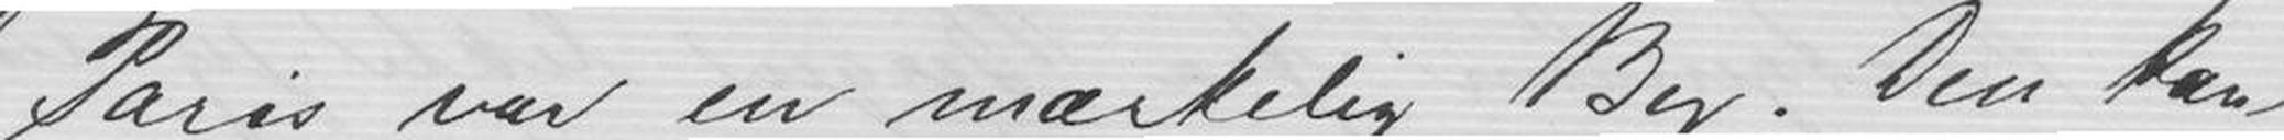Paris var en mærkelig By. Den kan
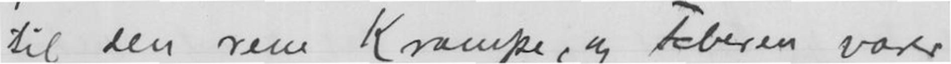til den rene Krampe, og Feberen varer
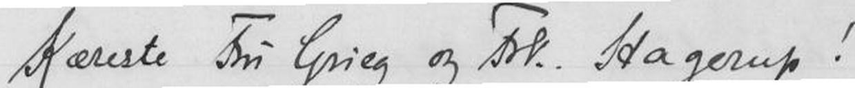Kæreste Fru Grieg og Frk. Hagerup!
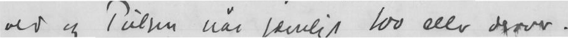ved og Pulsen når jævnlig 100 eller derover.
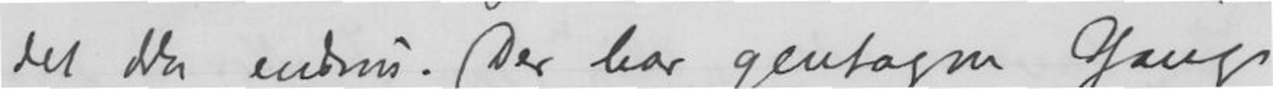det ikke endnu. Der har gentagne Gange
